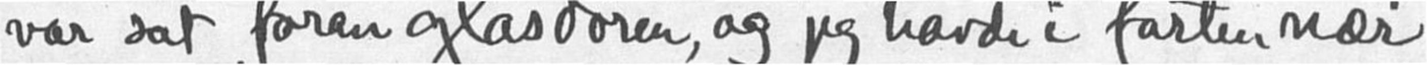var sat foran glasdøren, og jeg havde i farten nær
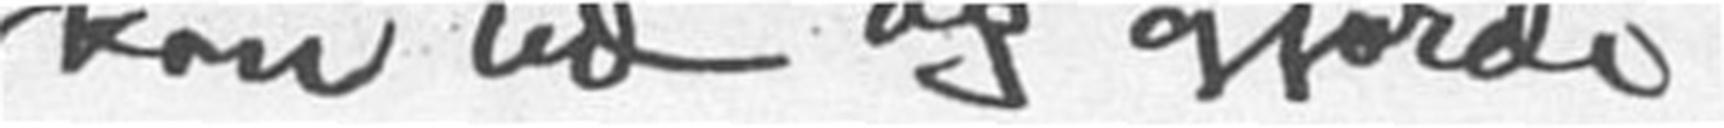kom ud og gjorde
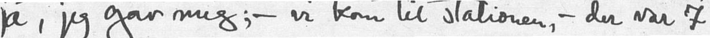ja, jeg gav mig; - vi kom til stationen, - der var 7
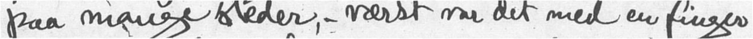paa mange steder,- værst var det med en finger
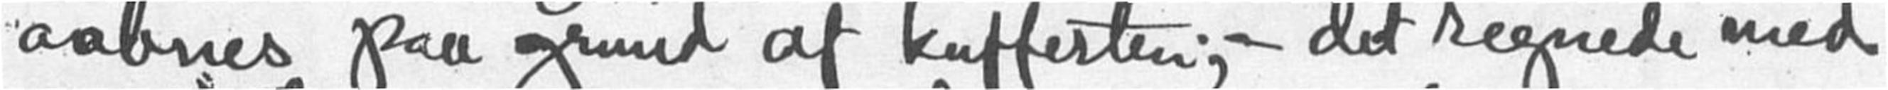aabnes paa grund af kufferten; - det regnede med
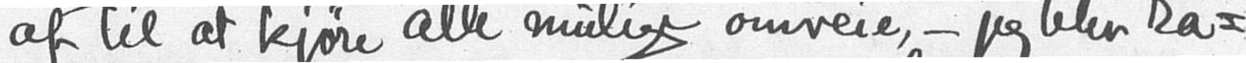af til at kjøre alle mulige omveie, - jeg blev ra-
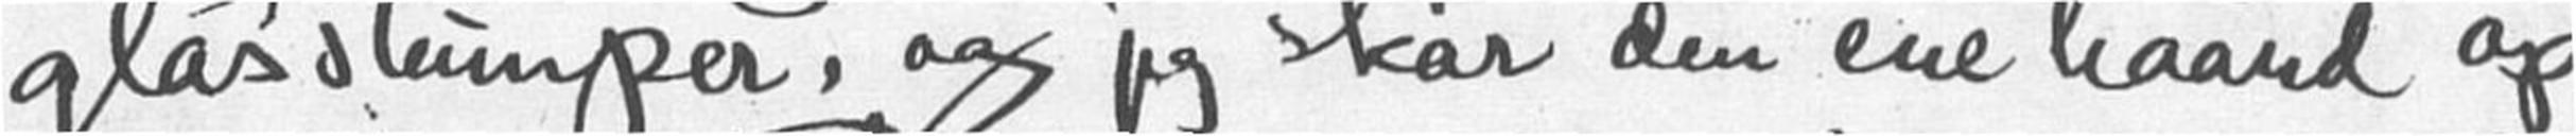glasstumper, og jeg skar den ene haand op
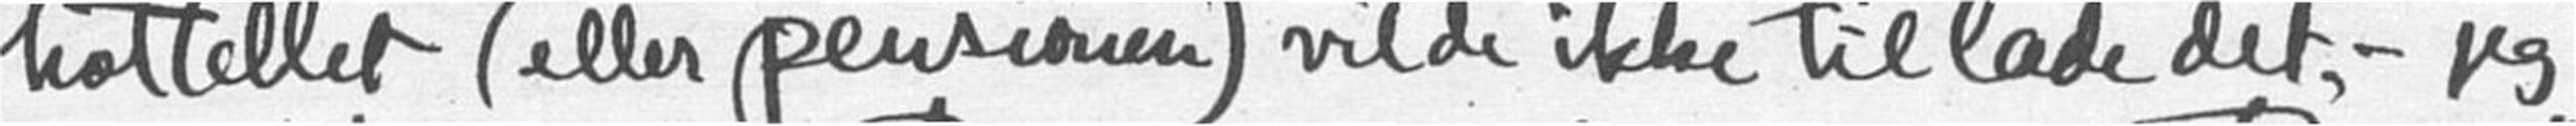hotellet (eller pensionen) vilde ikke tillade det, - jeg
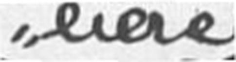"eiere"
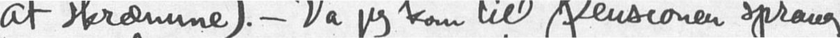at skræmme). - Da jeg kom til pensionen sprang
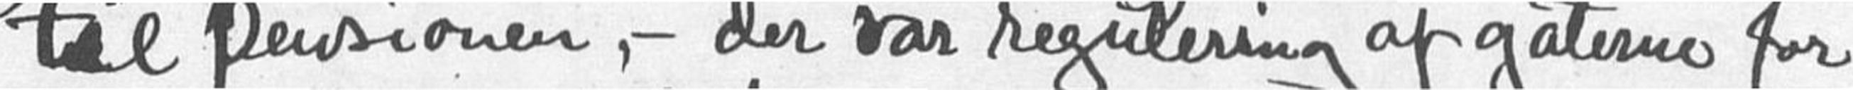til pensionen, - der var regulering af gaterne for
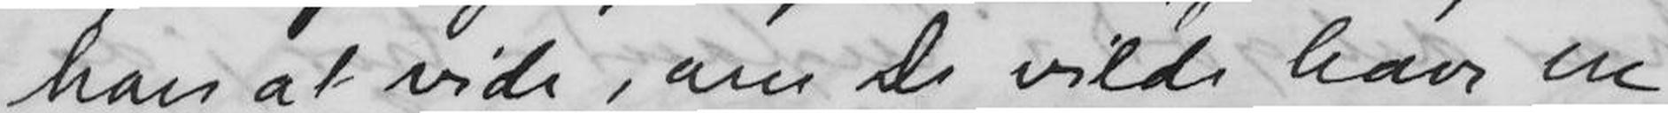have at vide, om De vilde have en
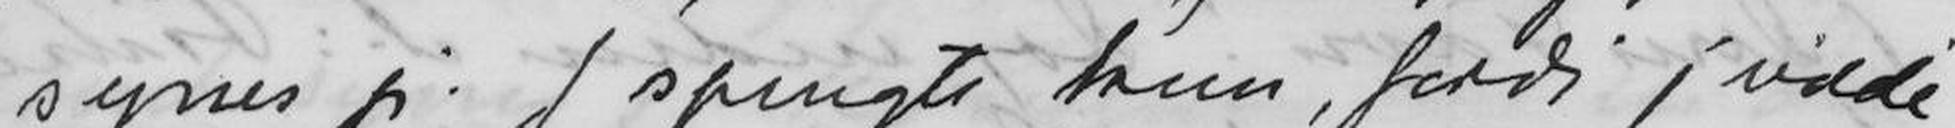synes jeg. J spurgte kun, fordi j vilde
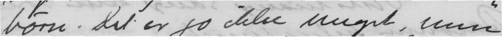børn. Det er jo ikke meget, men
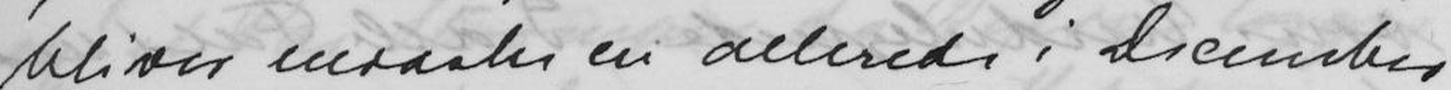bliver maaske en allerede i December.
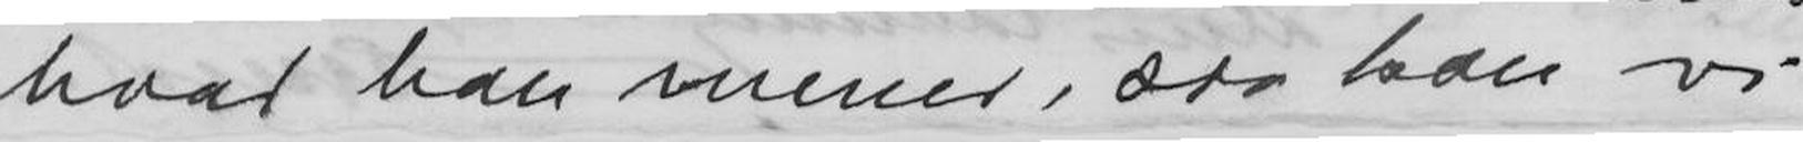hvad han mener, daa kan vi
